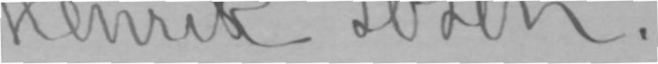Henrik Ibsen.
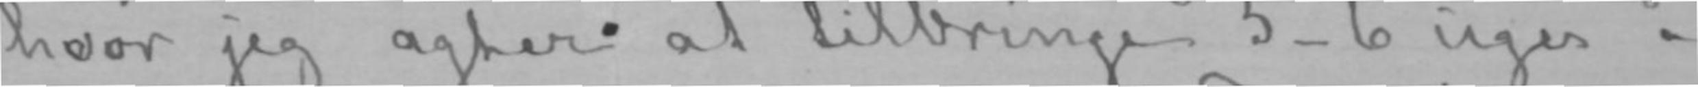hvor jeg agter at tilbringe 5-6 uger og
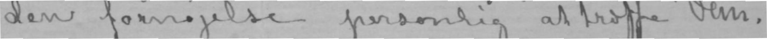den fornøjelse personlig at træffe Dem.
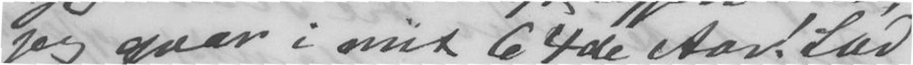jeg gaar i mit 64de Aar! Lad
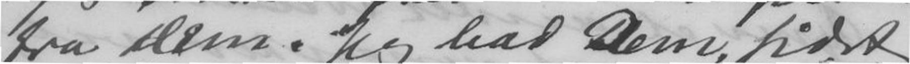fra Dem. Jeg bad Dem sidst
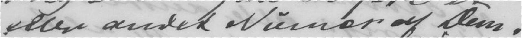eller andet Numer af Dem.
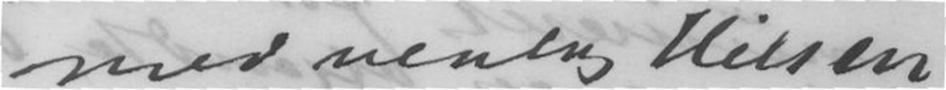med venlig Hilsen
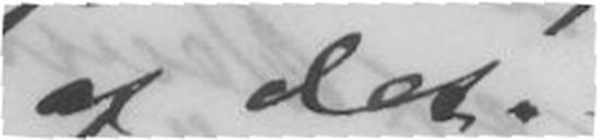af det.
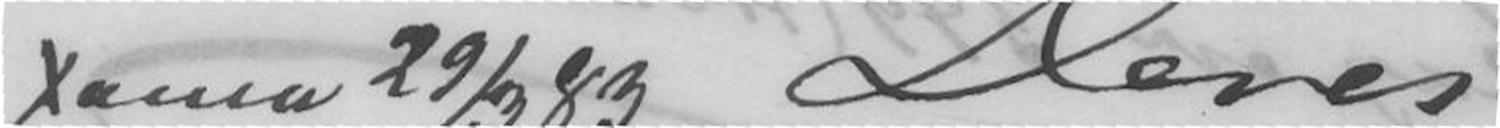Xania 26/3 83 Deres
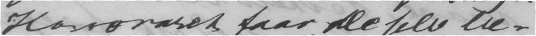Honoraret faar De selv Be-
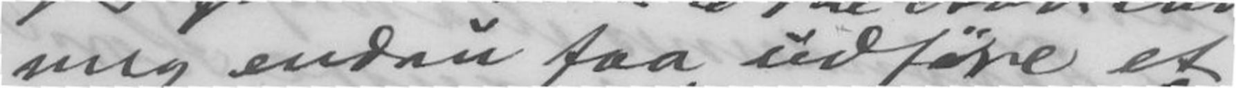mig endnu faa udføre et
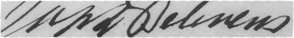Joh D Behrens
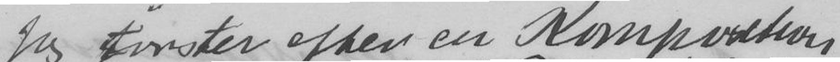Jeg tørster efter en Kompotion
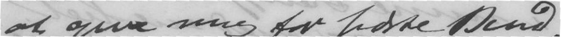at give mig for sidste Bind,
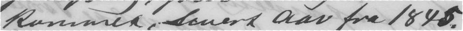kommet, hvert Aar fra 1845.
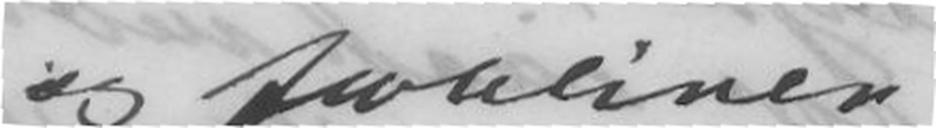og forbliver
